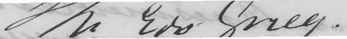Hr Edv Grieg.
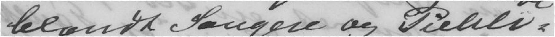blandt Sangere og Publi-
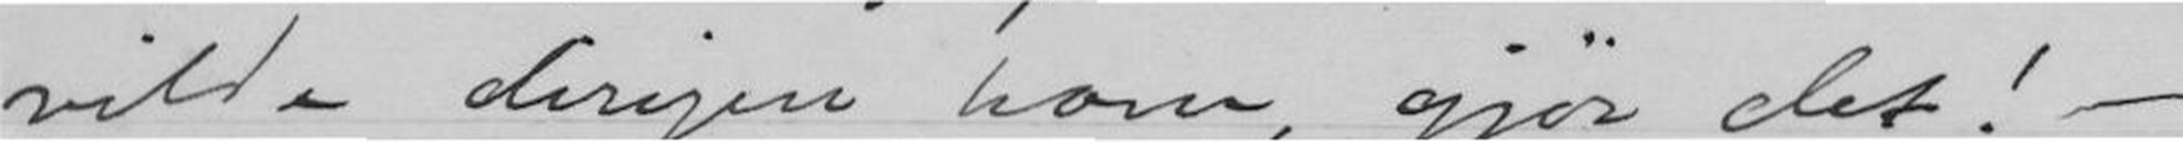vilde dirigere ham, gjør det! -
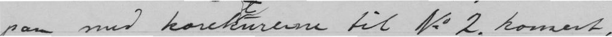paa med korekturerne til N. 2. konsert,
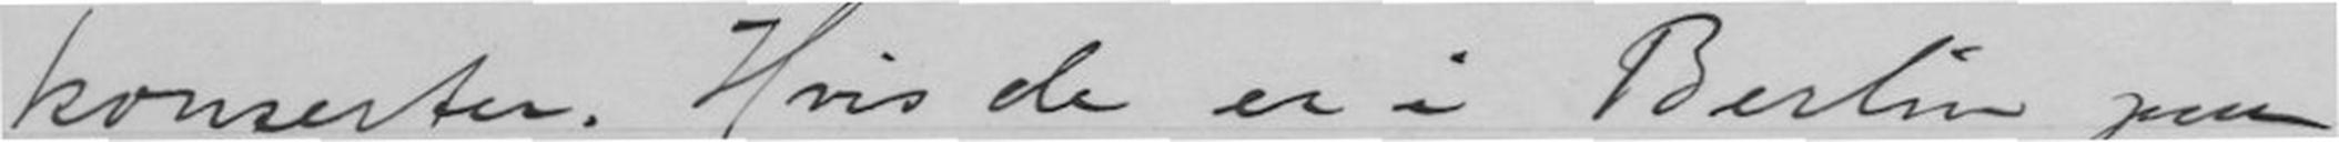konserter. Hvis de er i Berlin paa
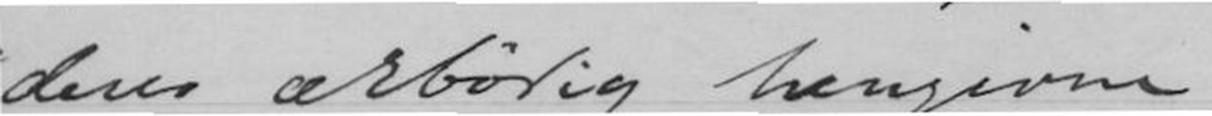deres ærbødig hengivne
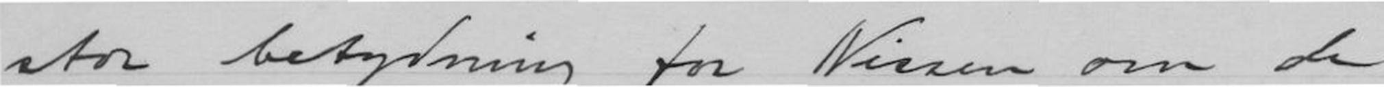stor betydning for Nissen om de
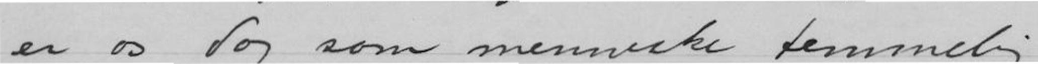er os dog som menneske temmelig
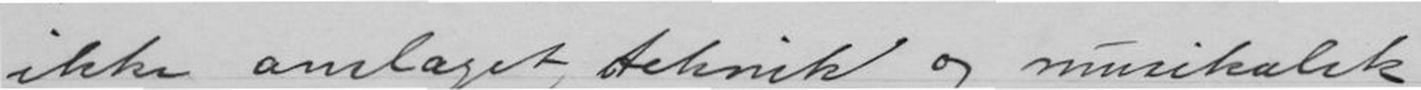ikke omlaget teknik og musikalsk
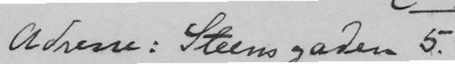Adresse: Steensgaden 5
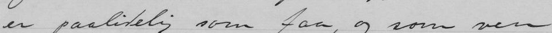er paalidelig som faa, og som ven
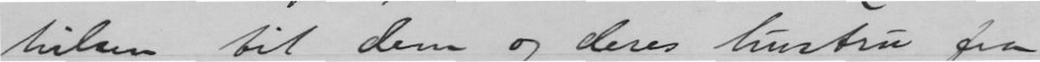hilsen til dem og deres hustru fra
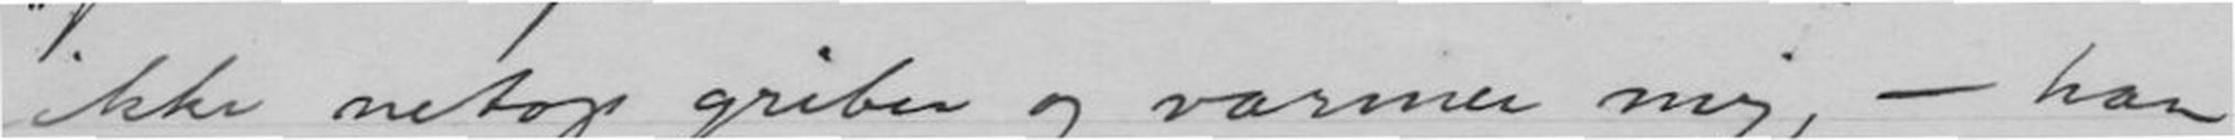ikke netop griber og varmer mig, - han
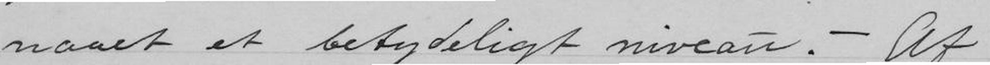naaet et betydeligt niveau. - Af
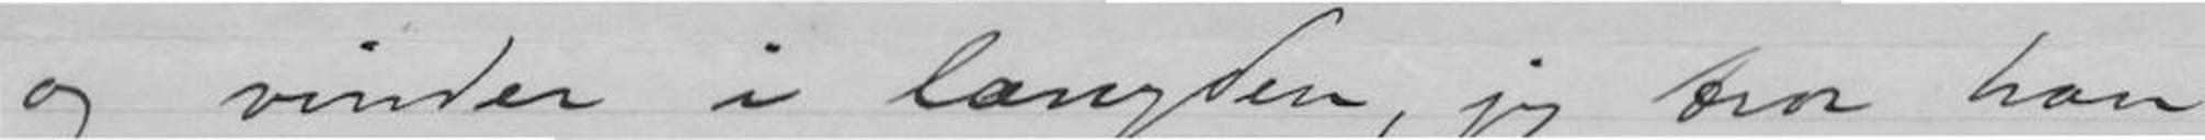og vinder i længden, jeg tror han
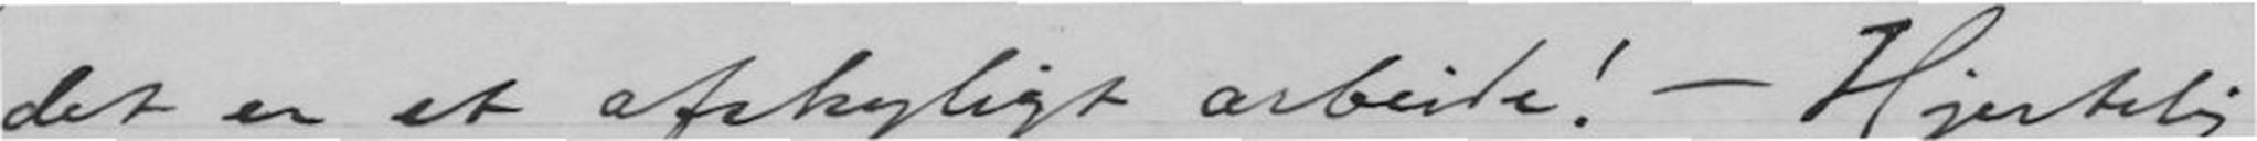det er et afskyligt arbeide! - Hjertelig
